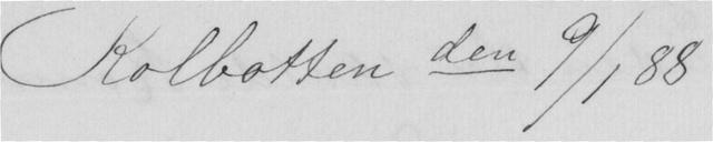Kolbotten den 9/1 88
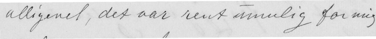alligevel, det var rent umulig for mig
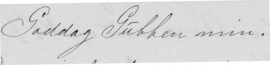Goddag Gubben min!
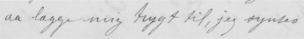aa lægge mig trygt til, jeg syntes
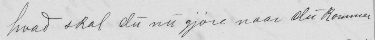hvad skal du nu gjøre naar du Kommer
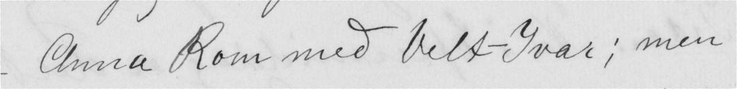- Anna Kom med belt-Ivar; men
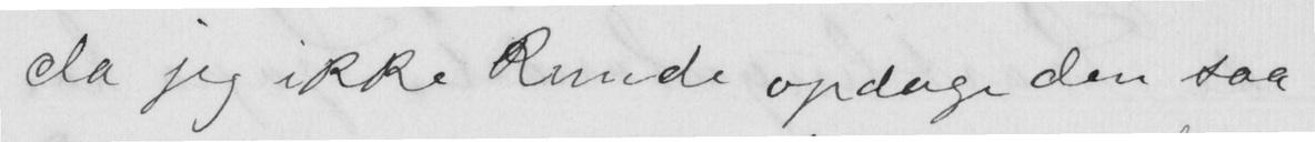da jeg ikke kunde opdage den saa
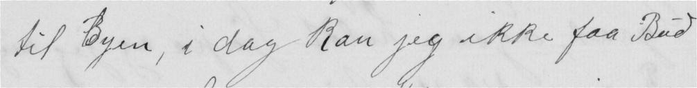til Byen, i dag kan jeg ikke faa Bud
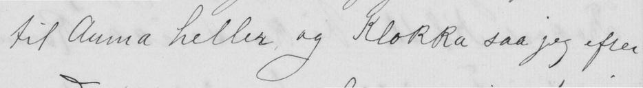til Anna heller og Klokka saa jeg efter
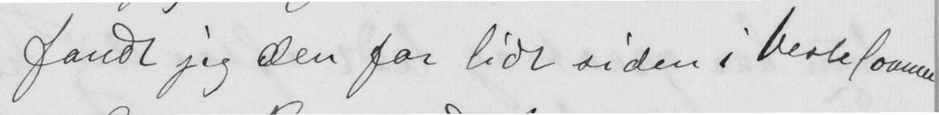fandt jeg den for lidt siden i Veolefomn
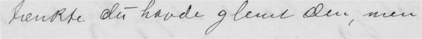tænkte du havde glemt den, men
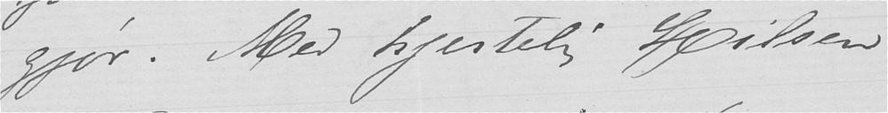gjør. Med hjertelig Hilsen
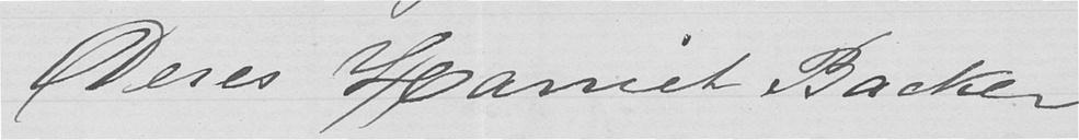Deres Harriet Backer
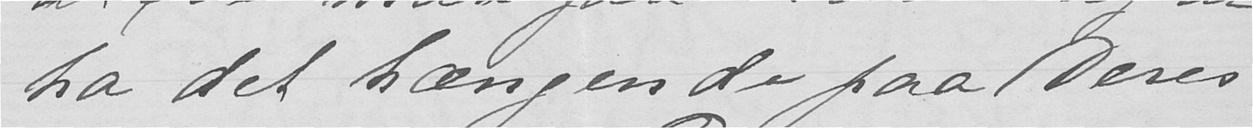ha det hængende paa Deres
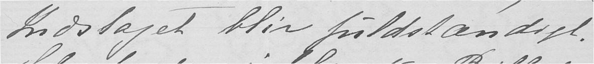Indslaget blir fuldstændigt.
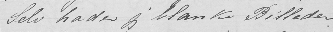Selv hader jeg blanke Billeder.
